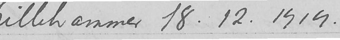Lillehammer 18.12.1919.
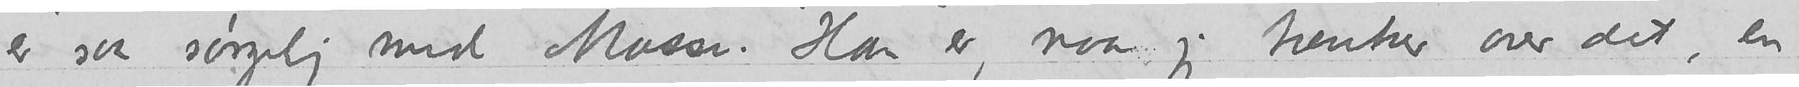er saa sørgelig med Mosse. Han er, naar jeg tænker over det, en
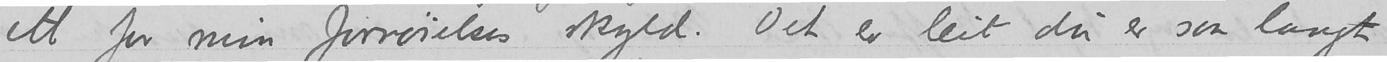ett for min fornøielses skyld. Det er leit du er saa langt
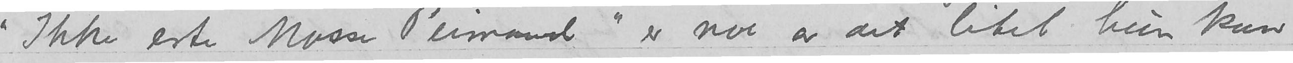«Ikke erte Mosse Peimand» er noe av det litet hun kan
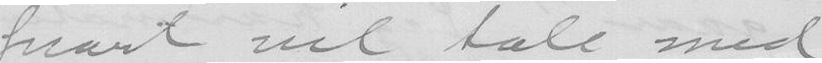snart vil tale med
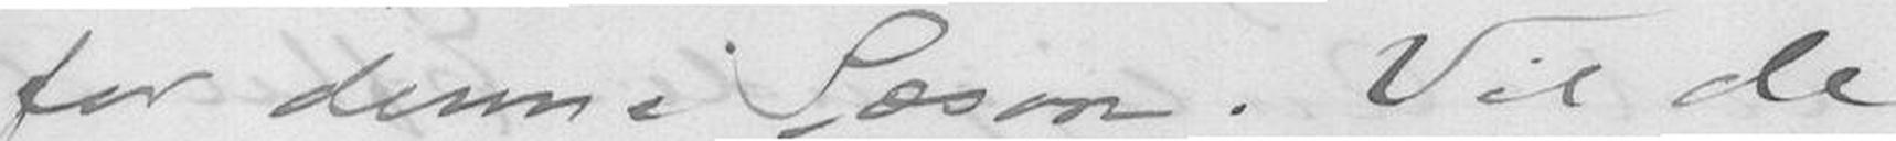for denne Sæson. Vil De
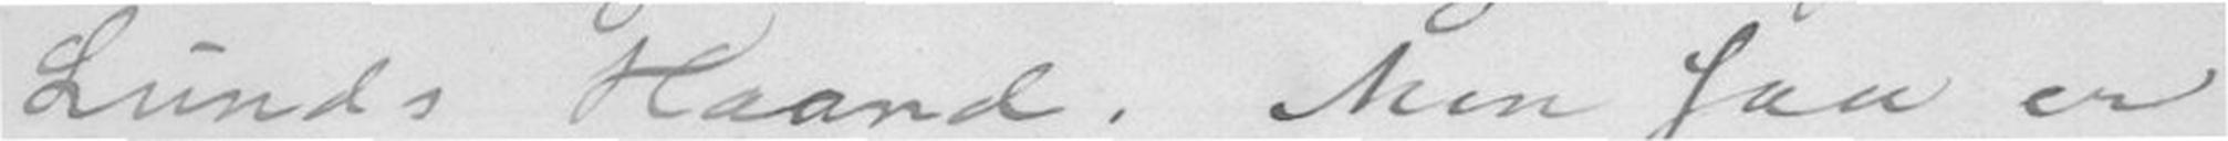Lunds Haand. Men saa er
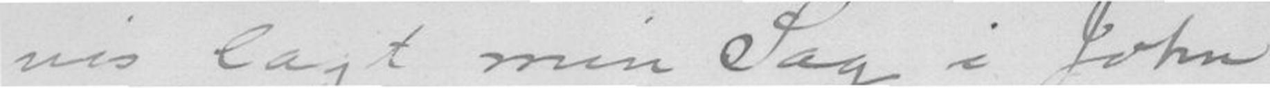vis lagt min Sag i John
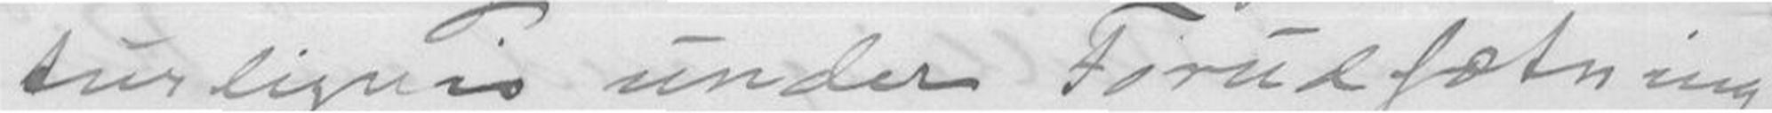turligvis under Forudsætning
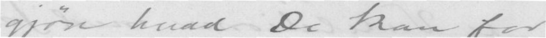gjøre hvad De kan for
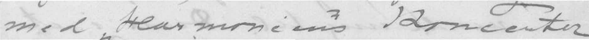med "Harmoniens" Koncerter
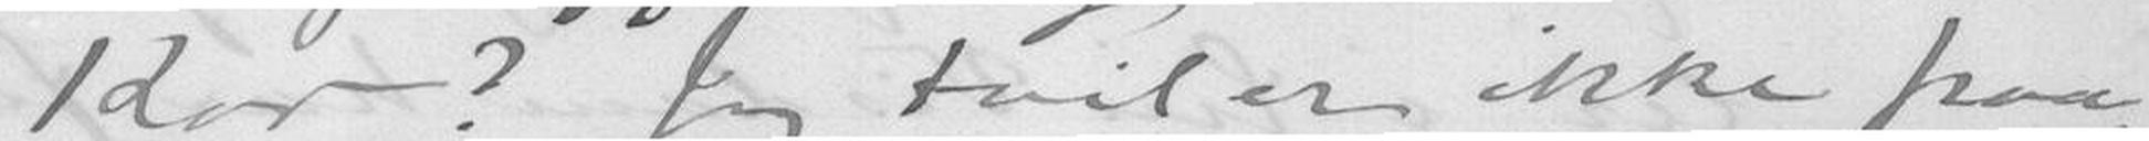Kor? Jeg tviler ikke paa,
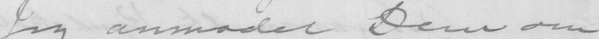Jeg anmoder Dem om
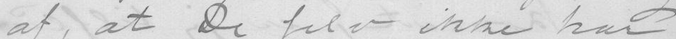af, at De selv ikke har
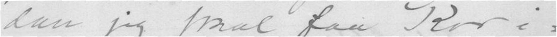dan jeg skal faa Kor i-
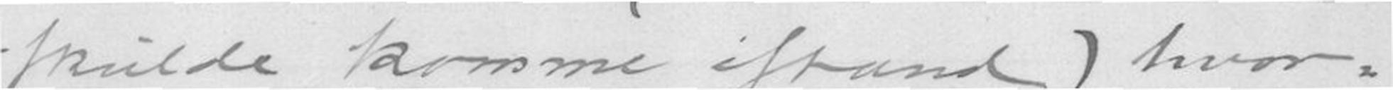skulde komme istand) hvor-
